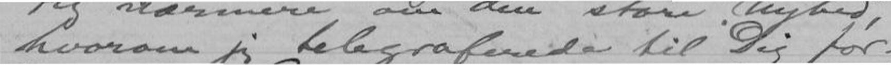hvorom jeg telegraferede til Dig for-
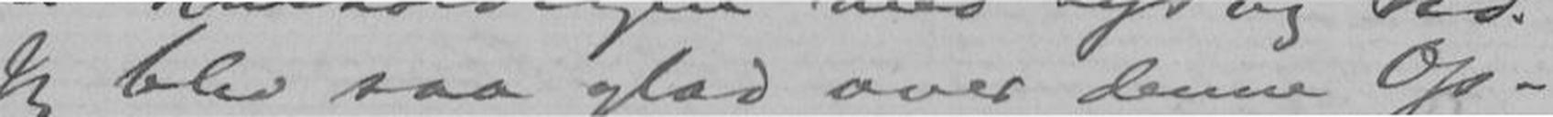Jeg blev saa glad over denne Op-
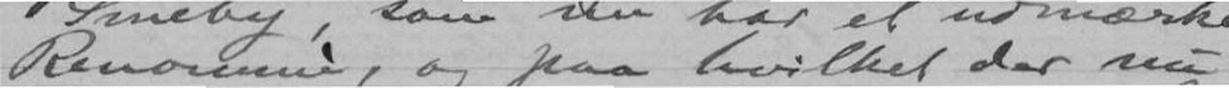Renommé, og paa hvilket der nu
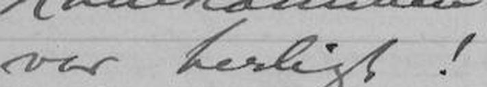var herligt!
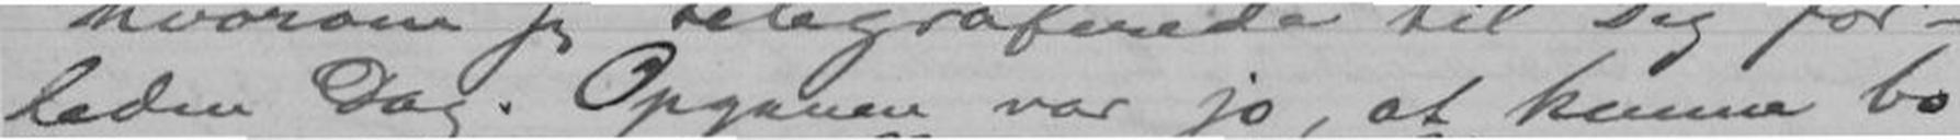leden Dag. Opgaven var jo, at kunne bo
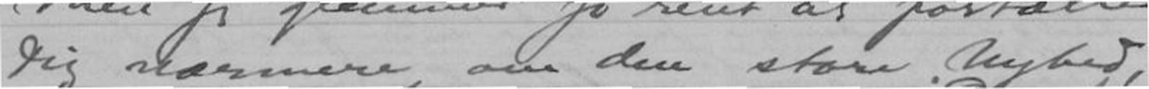Dig nærmere, om den store Nyhed,
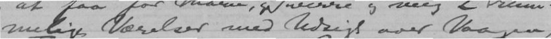melige Værelser med Udsigt over Vaagen,
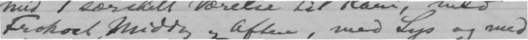Frokost, Middag og Aftens, med Lys og med
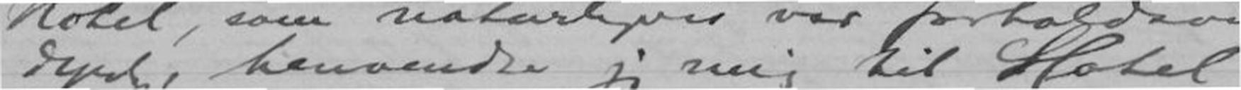dyrt, henvendte jeg mig til Hotel
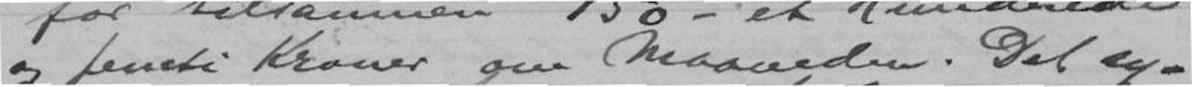og femti Kroner om Maaneden. Det sy-
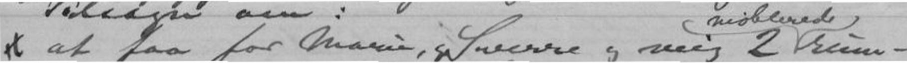at faa for Marie, Sverre og mig 2 rum-
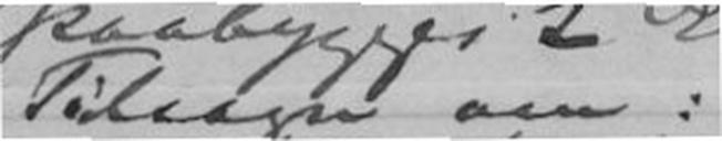Tilsagn om:
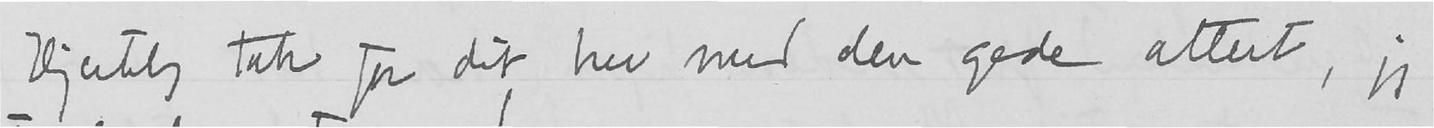Hjertelig tak for dit brev med den gode attest, jeg
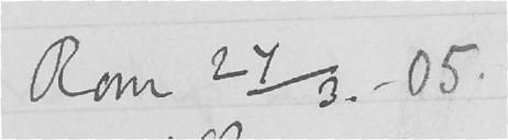Rom 27/3. – 05
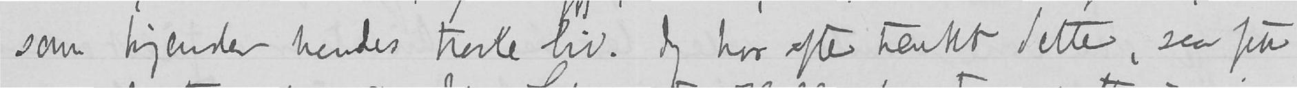som kjender hendes travle liv. Jeg har ofte tænkt dette, saa fik
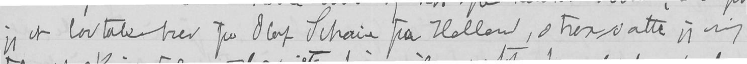jeg et lovtalebrev fra Olaf Schou fra Holland, strax satte jeg mig
